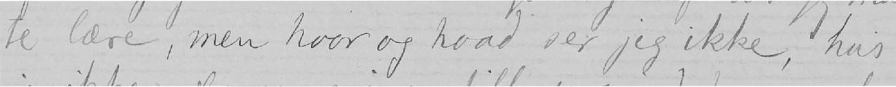te lære, men hvor og hvad ser jeg ikke, hvis
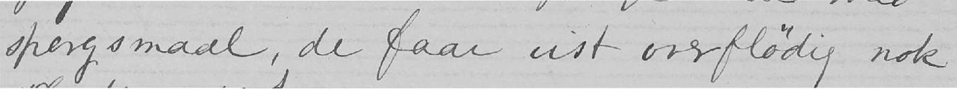spørgsmaal, de faar vist overflødig nok
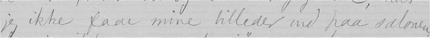jeg ikke faar mine billeder ind paa salonen,
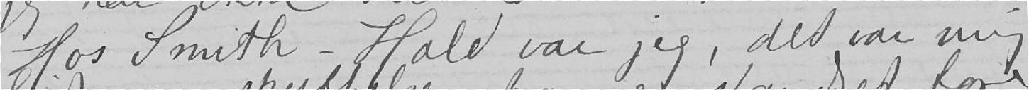Hos Smith-Hald var jeg, det var mig
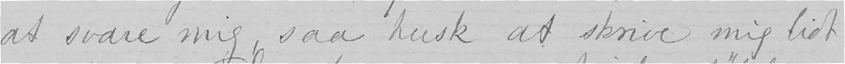at svare mig, saa husk at skrive mig lidt
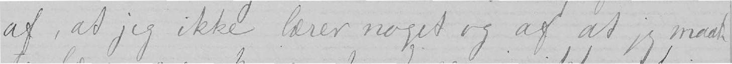af, at jeg ikke lærer noget og af at jeg maat-
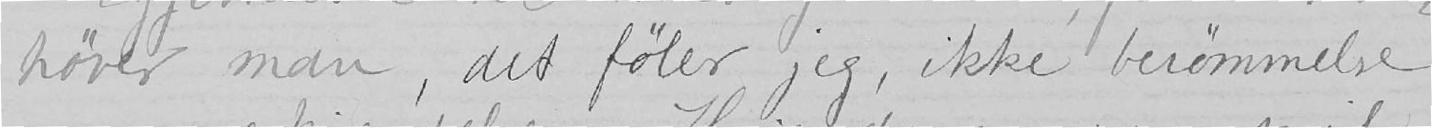høver man, det føler jeg, ikke berømmelse
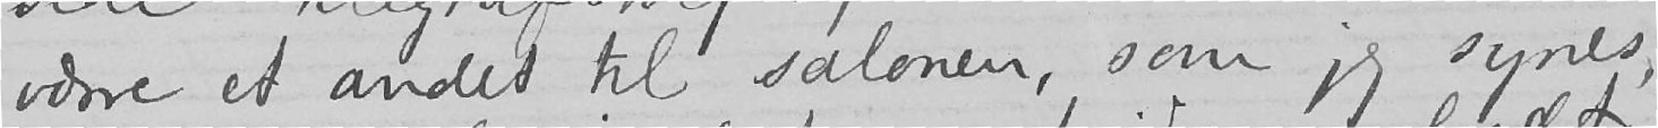værre et andet til salonen, som jeg synes,
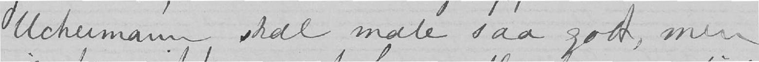Uchermann skal male saa godt, men
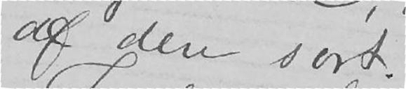af den sort.
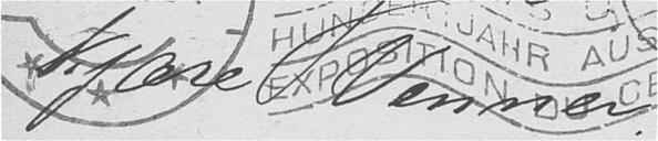kjære Venner!
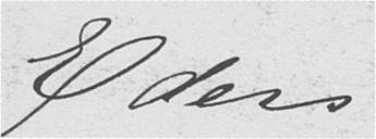Eders
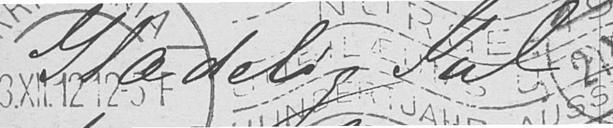Glædelig Jul
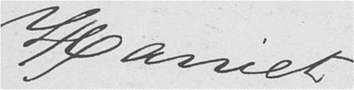Harriet
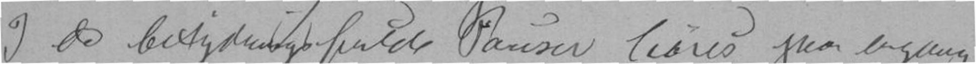I de betydningsfulde Pauser høres paa engang
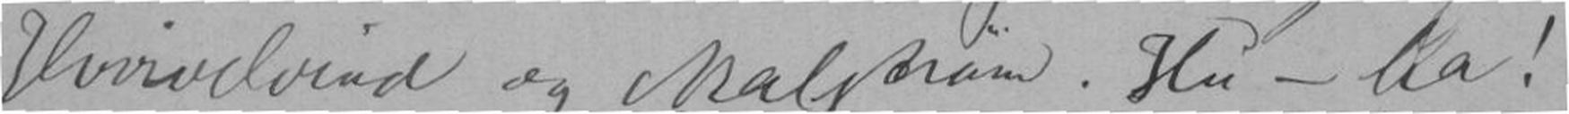Hvirvelvind og Malstrøm. Hu - ha!
